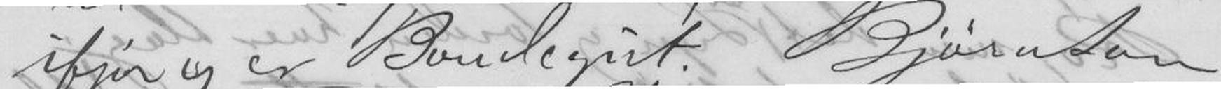ifjor og er Bondegut, Bjørnson
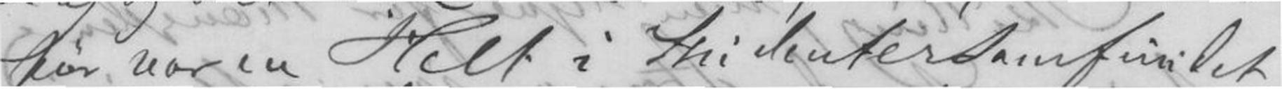før var en Helt i Studentersamfundet
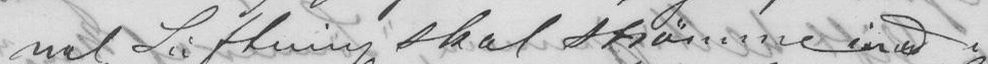nal Luftning skal Strømme ind i
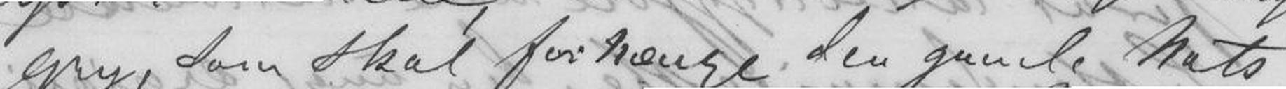gry, den skal fortrænge den gamle Nats
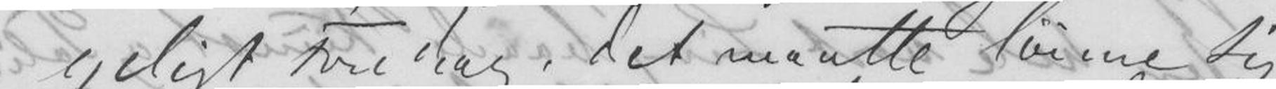geligt Foredrag, det maatte lønne sig
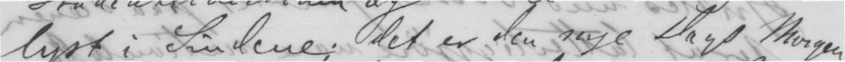lyst i Sindene; det er den nye Dags Morgen
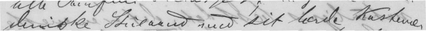demiske Stueaand med sit lærde Kastevæ-
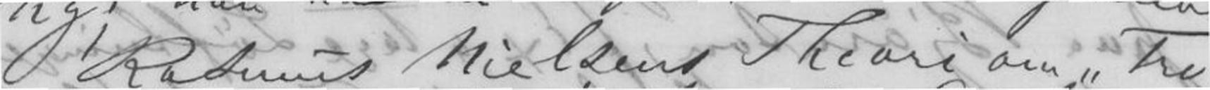Rasmus Nielsens Theori om Tre
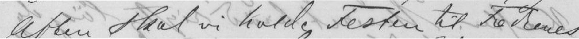Aften skal vi holde Festen til Fædrenes
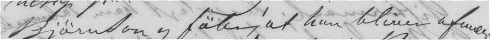Bjørnson og føler, at han bliver afmeg-
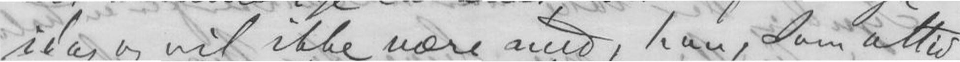idag og vil ikke være med, han, som altid
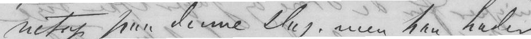netop paa denne Dag, men han hader
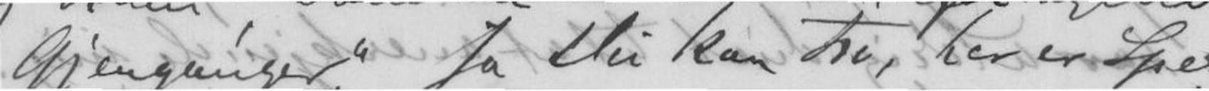Gjenganger. Ja Du kan Tro, her er Spe
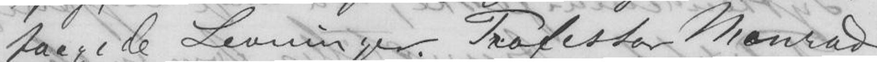fargede Levninger; Professor Manrad,
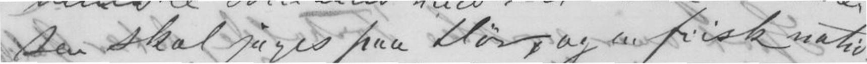sen skal jages paa Dør, og en frisk natio-
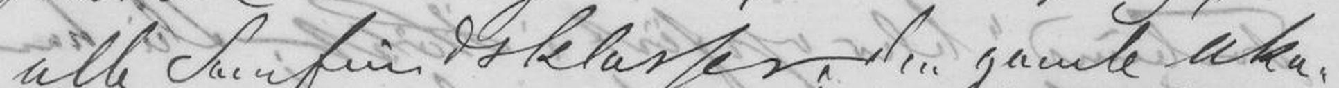alle Samfundsklasser; den gamle aka-
